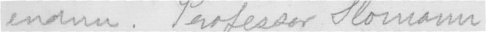endnu. Professor Slomann
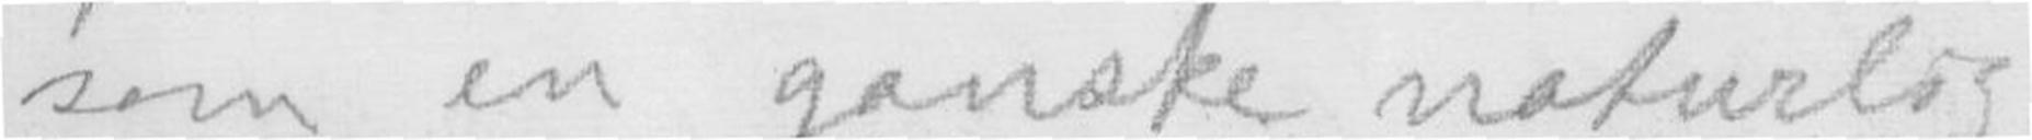som en ganske naturlig
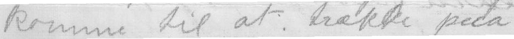komme til at trække paa
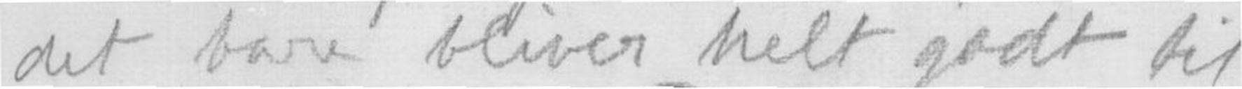det bare bliver helt godt til
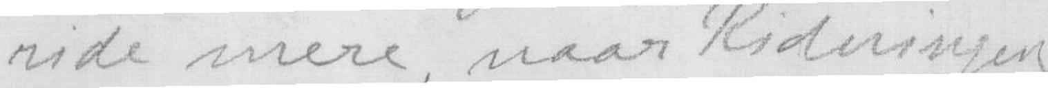ride mere, naar Ridningen
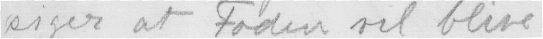siger at Foden vil blive
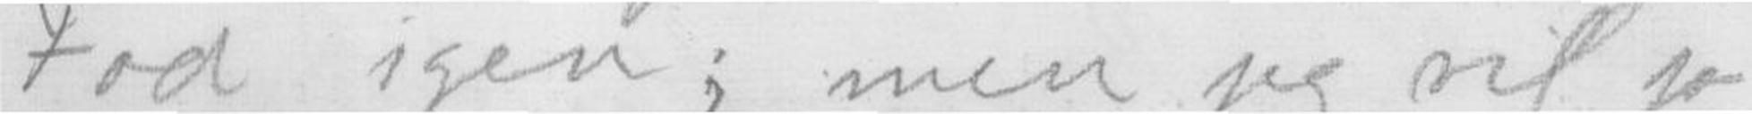Fod igen; men jeg vil jo
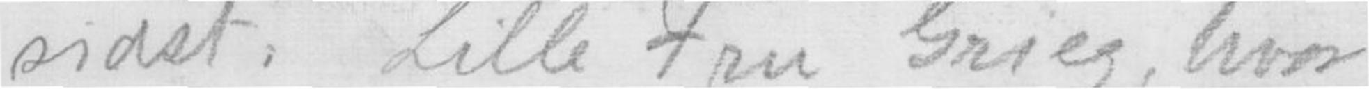sidst. Lille Fru Grieg, hvor
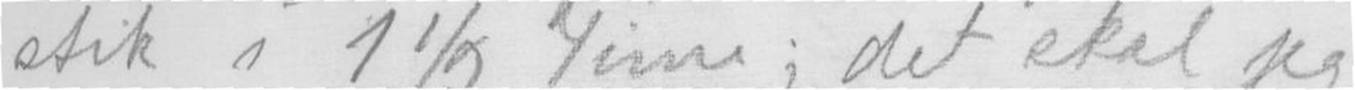stik i 1 1/2 Time; det skal jeg
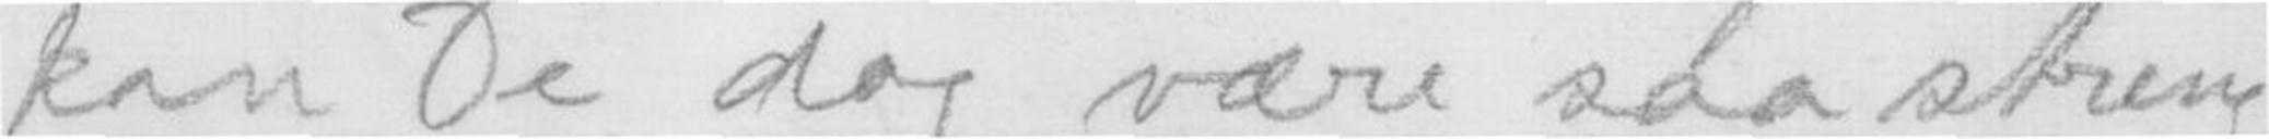kan De dog være saa streng
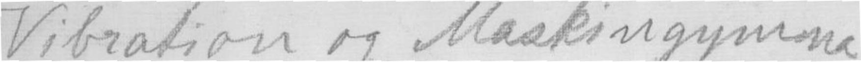Vibration og Maskingymna
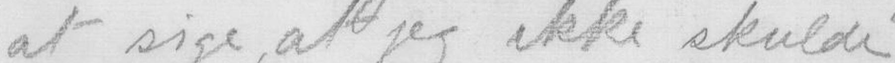at sige, at jeg ikke skulde
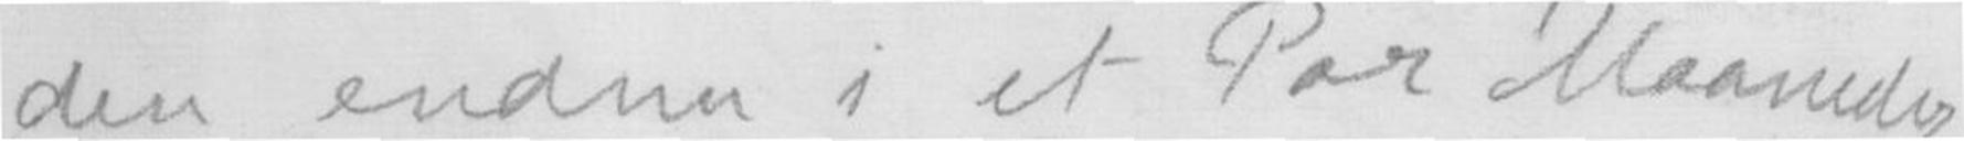den endnu i et Par Maaneder
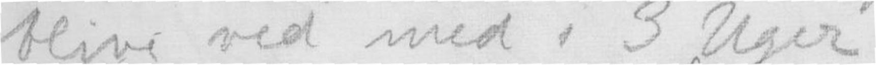blive ved med i 3 Uger
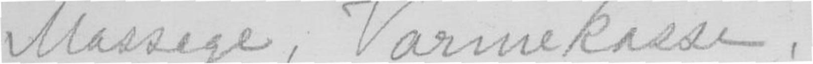Massage, Varmekasse,
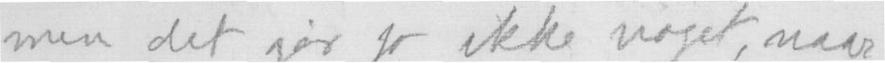men det gjør jo ikke noget, naar
